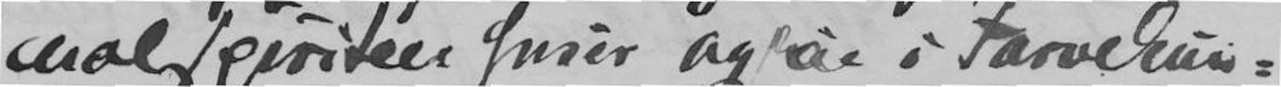nalspiriten suser og saa i Farvekun-
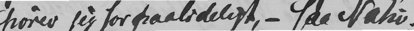hører jeg for paalideligt, - saa Natio-
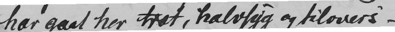har gaat her træt, halvsyg og tilovers -
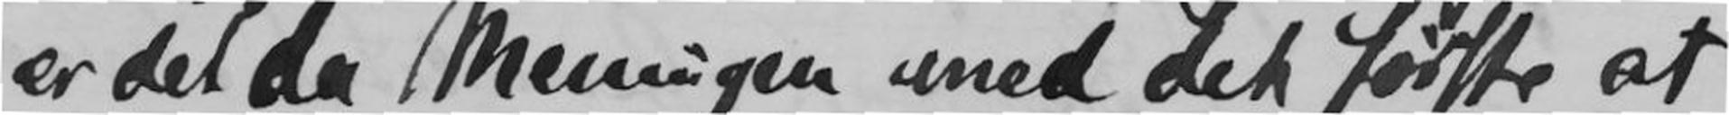er det da Meningen med det første at
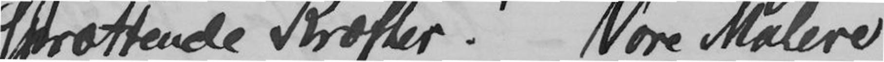Sprættende Kræfter. Vore Malere
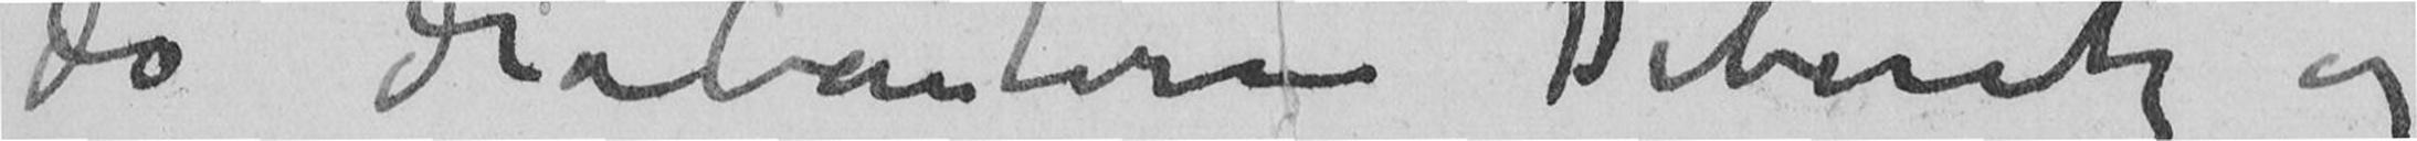da drabanterne Deberitz og
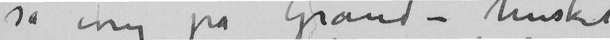så enig på Grand – husket
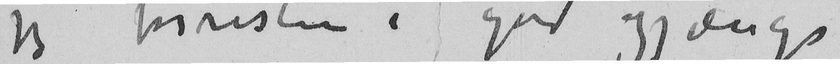jeg forresten i god gjænge
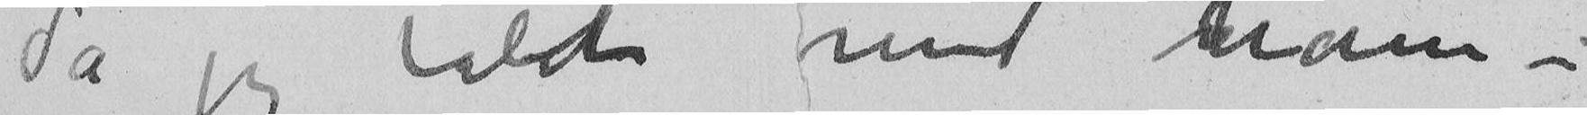da jeg talte med ham –
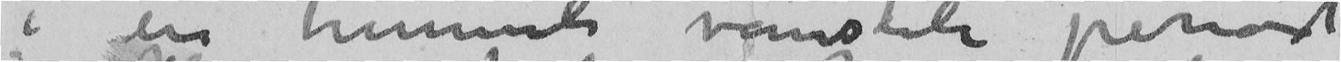i en temmeli vanskeli periode
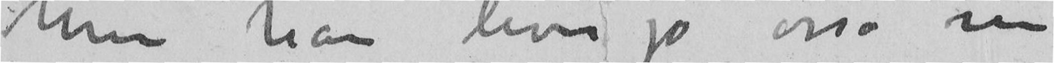Men han lever jo osså nu
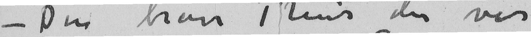– Den brave Thiis der var
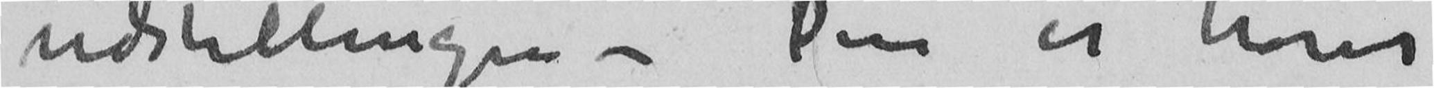udstillingen – Den er hører
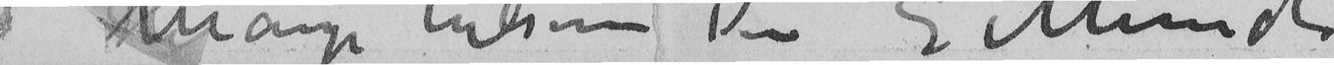HMange hilsener Din E Munch
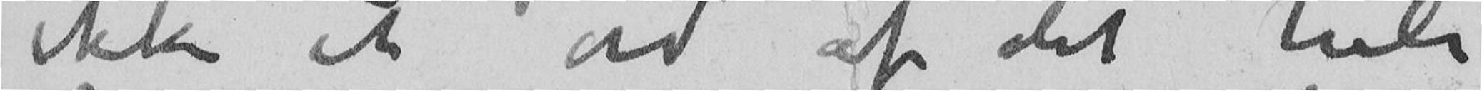ikke et ord av det hele
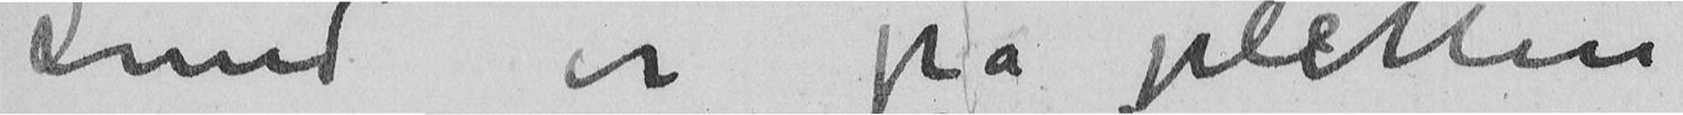Lund er på pletten
